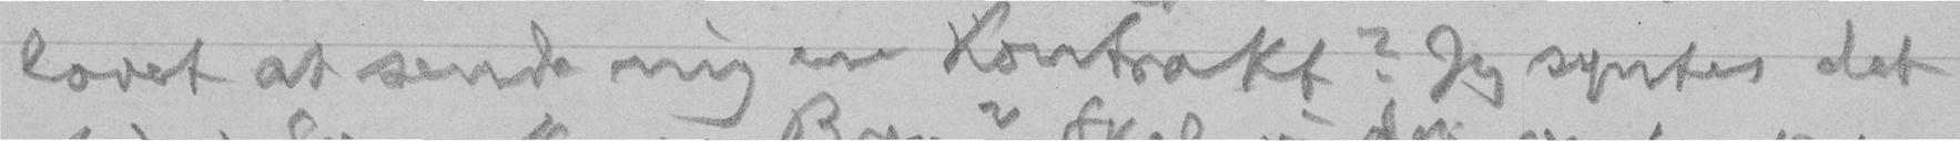lovet at sende mig en Kontrakt? Jeg syntes det
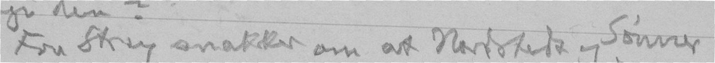Fru Stray snakker om at Nordstedt og Sønner
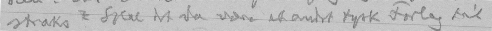straks? Skal det da være et andet tysk Forlag til
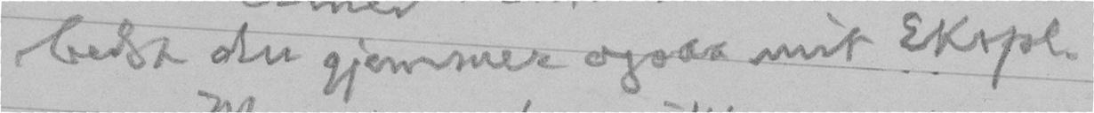bedst du gjemmer ogsaa mit Ekspl.
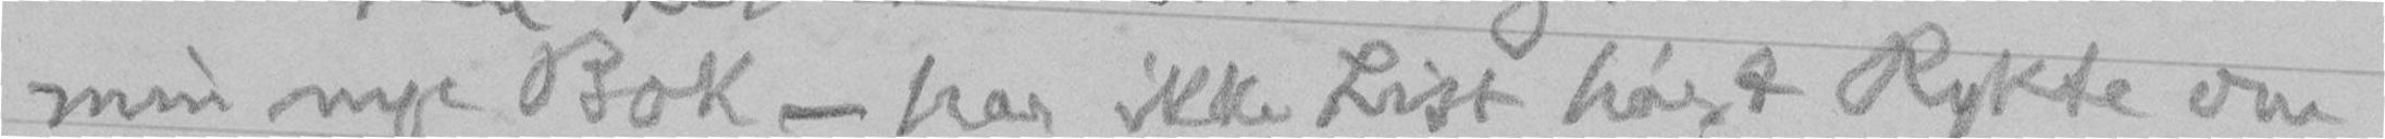min nye Bok - har ikke List hørt Rykte om
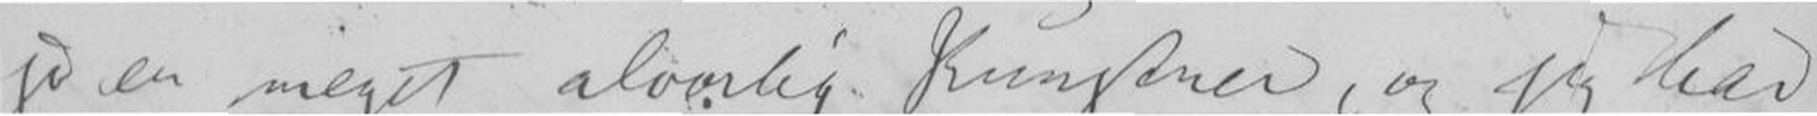jo en meget alvorlig Kunstner, og jeg har
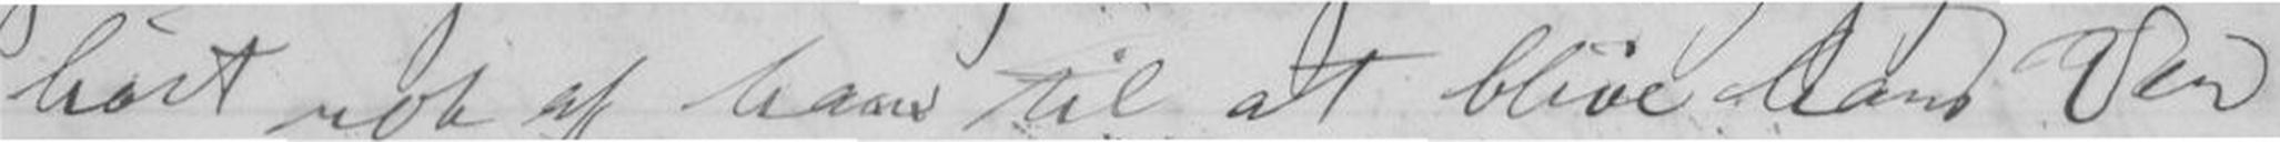hørt nok af ham til at blive hans Ven
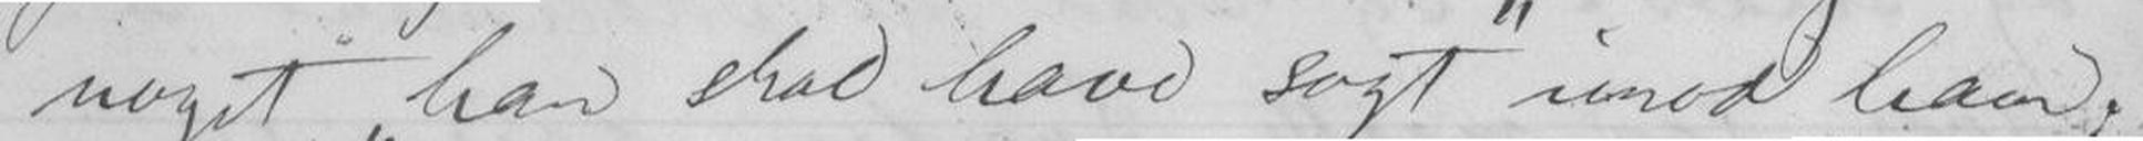noget han skal have sagt imod ham;
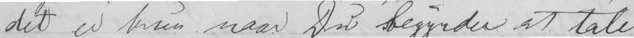det er kun naar Du begynder at tale
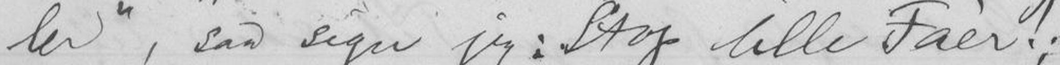ler, saa siger jeg: Stop lille Faér!;
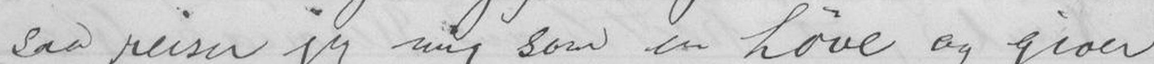saa reiser jeg mig som en Løve og giver
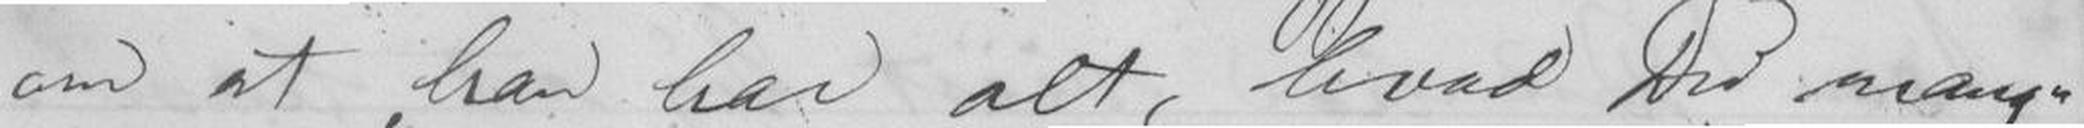om at han har alt, hvad Du mang-
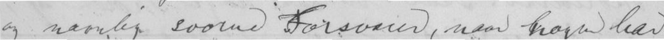og navnlig svorne Forsvarer, naar nogen har
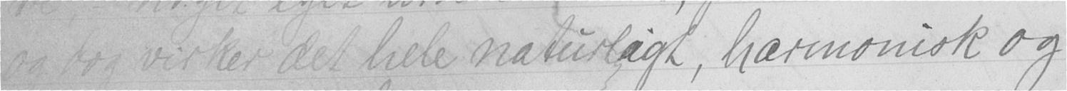og dog virker det hele naturligt, harmonisk og
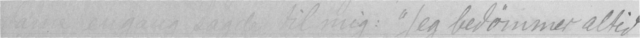engang sagde til mig: "Jeg bedømmer altid
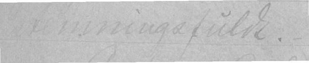stemningsfuldt. -
